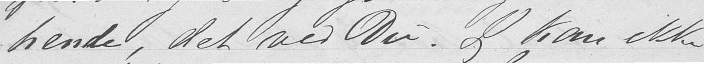hende, det ved Du. Jeg kan ikke
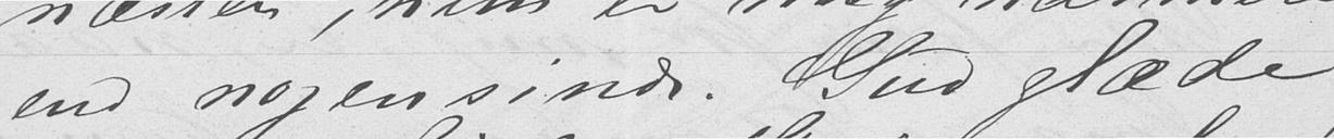end nogensinde. Gud glæde
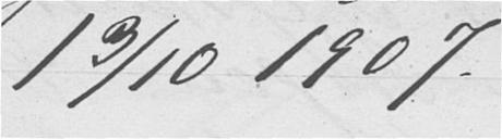13/10 1907.
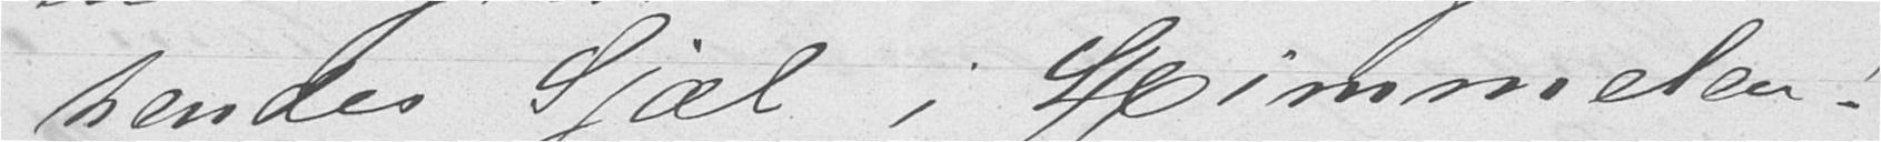hendes Sjæl i Himmelen!
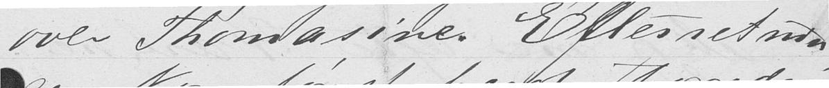over Thomasine. Efterretnin-
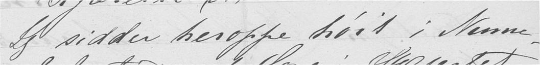Jeg sidder heroppe høit i Nume-
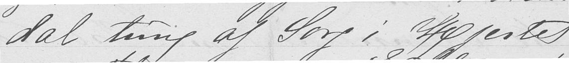dal tung af Sorg i Hjertet
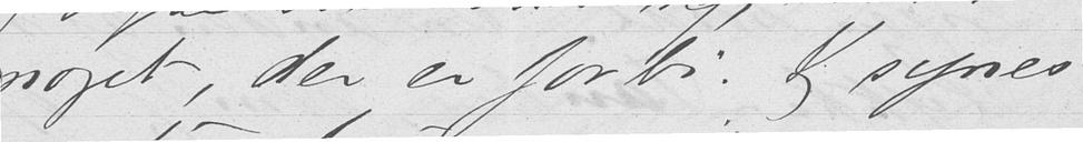noget, der er forbi. Jeg synes
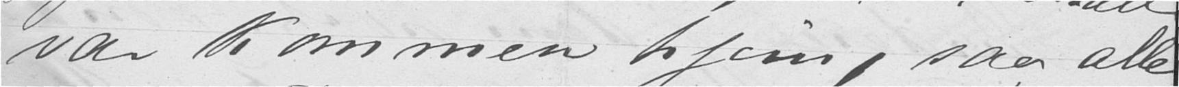var kommen hjem, saa alle
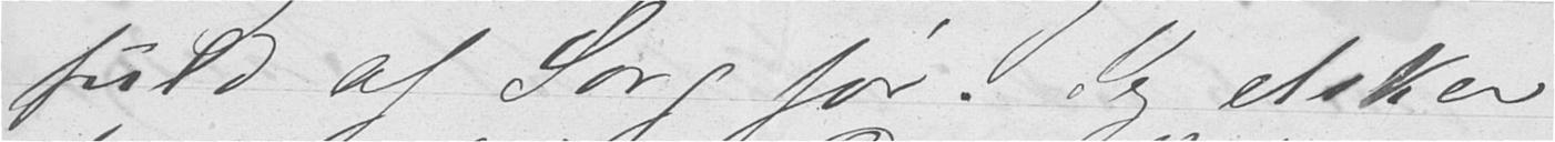fuld af Sorg før. Jeg elsker
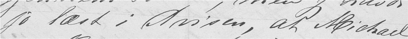jo læst i Avisen, at Michael
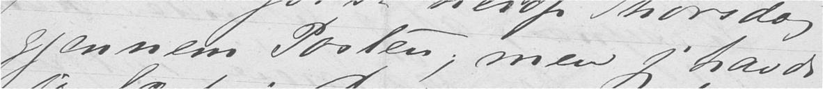gjennem Posten; men jeg havde
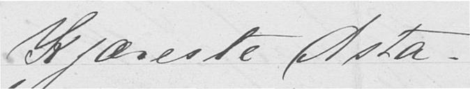Kjæreste Asta.
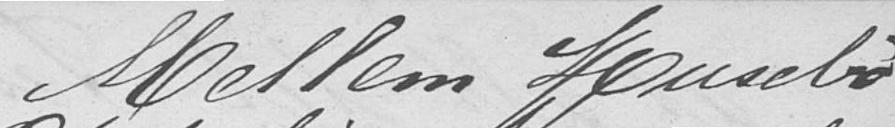Mellem Husebø
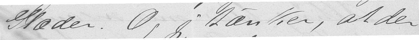Glæder! Og jeg tænker, at der
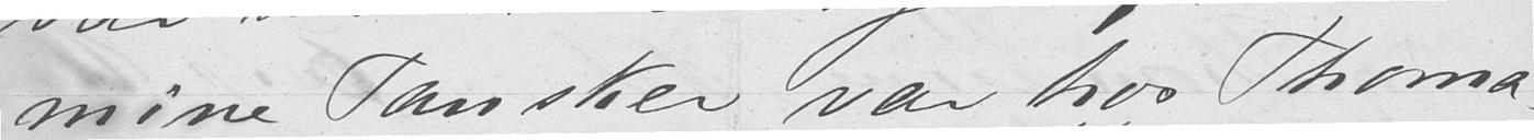mine Tansker var hos Thoma-
